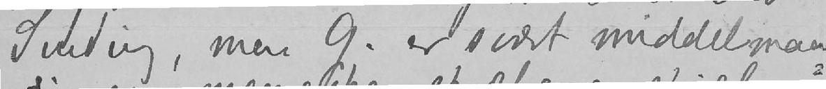Sinding, men G. er svært middelmaa-
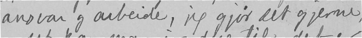ansvar og arbeide, jeg gjør det gjerne,
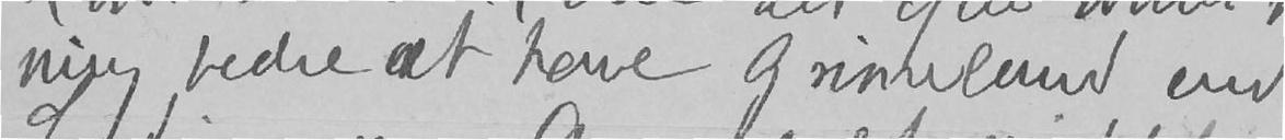ning bedre at have Grimelund end
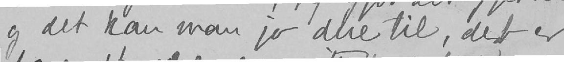og det kan man jo due til, det er
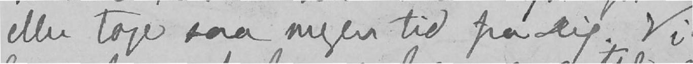eller tage saa megen tid fra Dig. Vi
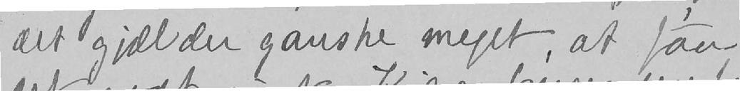det gjælder ganske meget, at faa
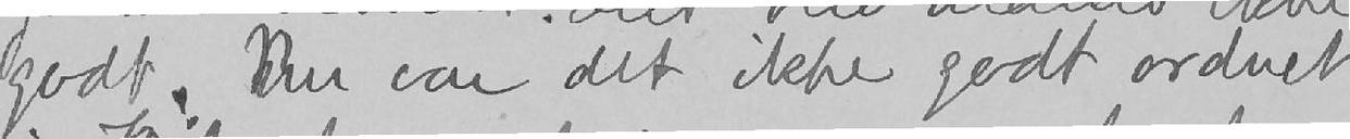godt. Nu var det ikke godt ordnet
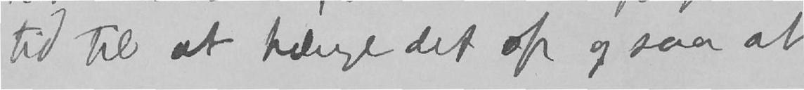tid til at hænge det op og saa at
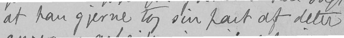at han gjerne tog sin part af dette
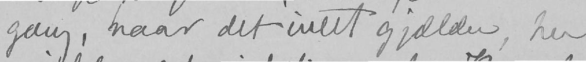gang, naar det intet gjælder, her
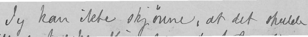Jeg kan ikke skjønne, at det skulde
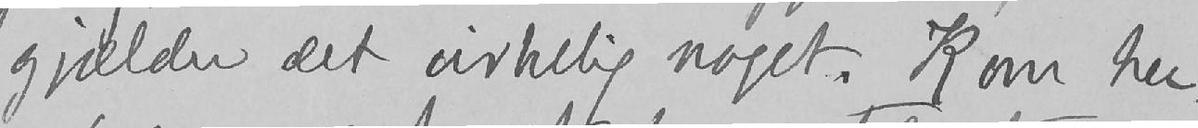gjælder det virkelig noget. Kom her-
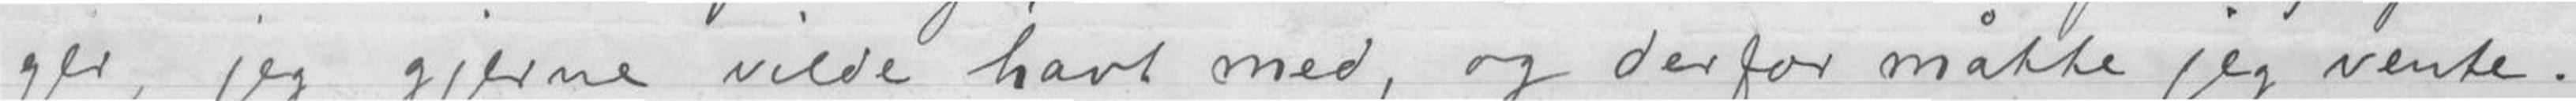ger, jeg gjerne vilde have med, og derfor måtte jeg vente.
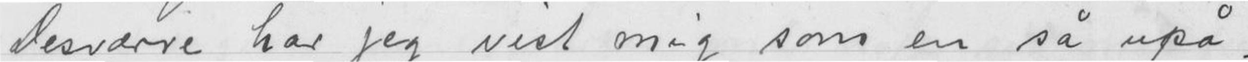Desværre har jeg vist mig som en så upå-
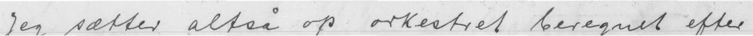Jeg sætter altså op orkesteret beregnet efter
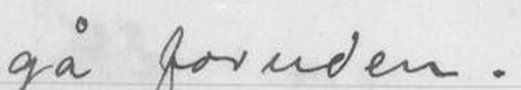gå foruden.
